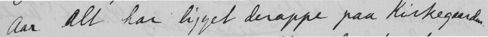aar alt har ligget deroppe paa Kirkegaarden.
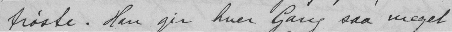trøste. Han gir hver Gang saa meget
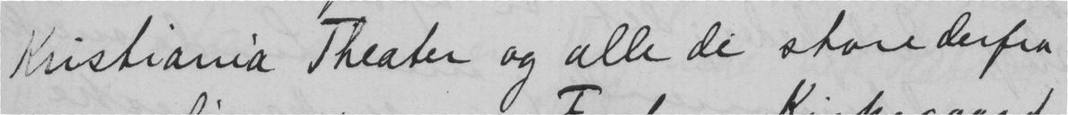Kristiania Theater og alle de store derfra
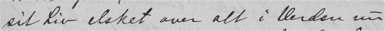sit Liv elsket over alt i verden nu
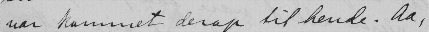var kommet derop til hende. Aa,
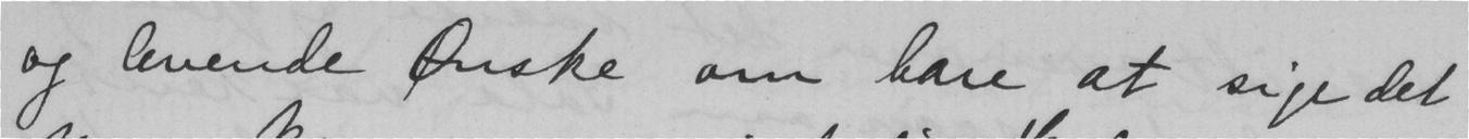og levende Ønske om bare at sige det
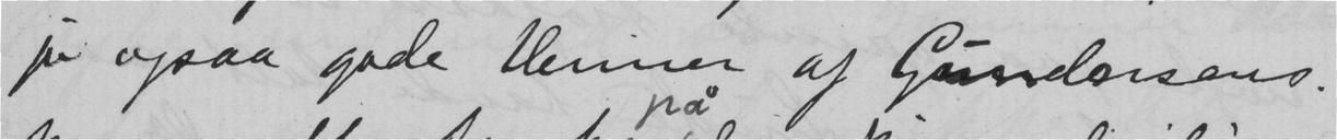jo ogsaa gode venner af Gundersens.
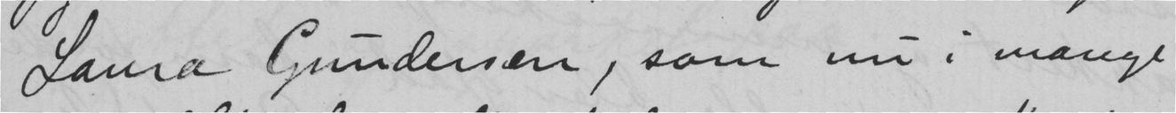Larna Gundersen, som nu i mange
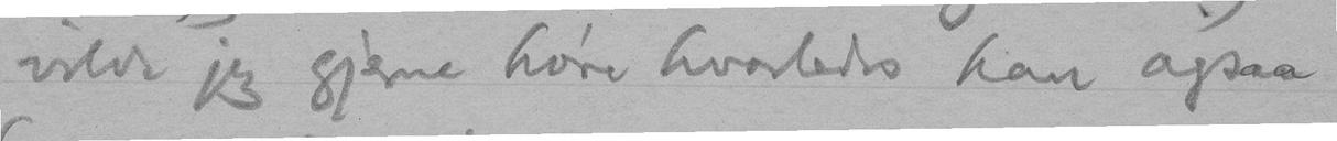vilde jeg gjerne høre hvorledes han ogsaa
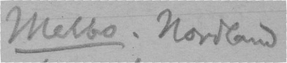Melbo. Nordland
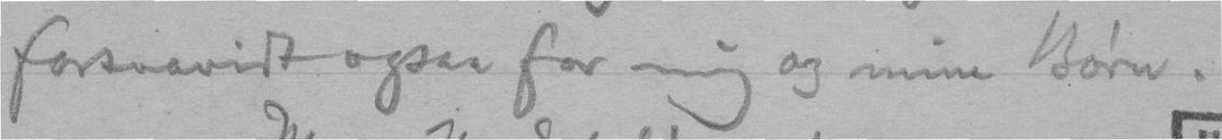forsaavidt ogsaa for mig og mine Børn.
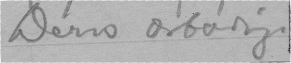Deres ærbødige
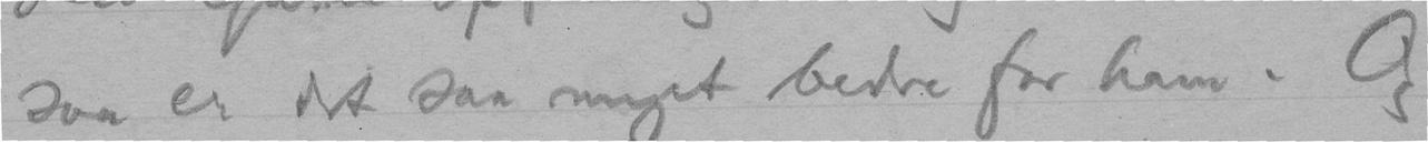saa er det saa meget bedre for ham. Og
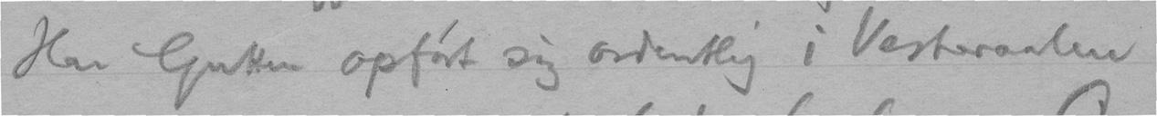Har Gutten opført sig ordentlig i Vesteraalen
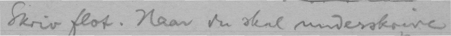Skriv flot. Naar du skal underskrive
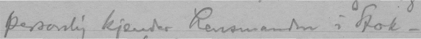personlig kjender Lensmanden i Stok-
